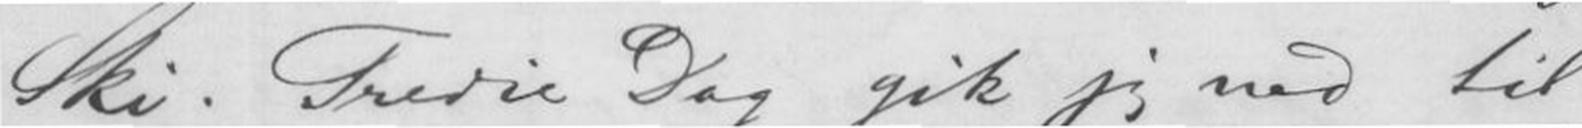Ski. Tredie Dag gik jeg ned til
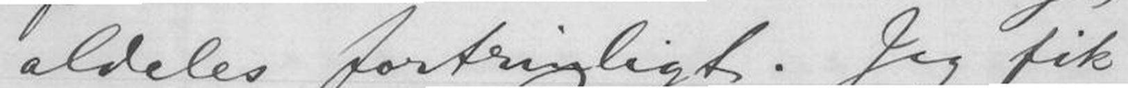aldeles fortrinligt. Jeg fik
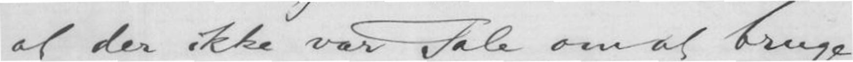at der ikke var Tale om at bruge
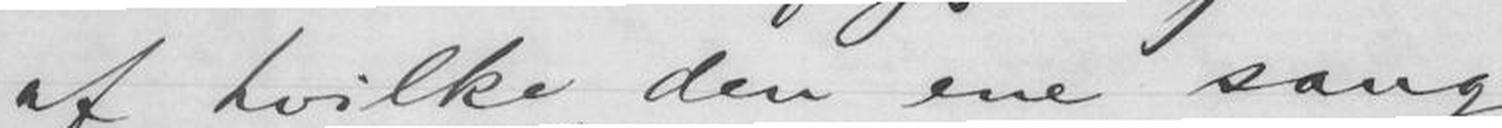af hvilke den ene sang
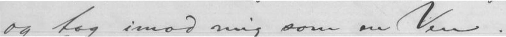og tog imod mig som en Ven.
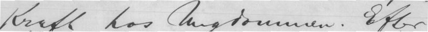Kraft hos Ungdommen. Efter
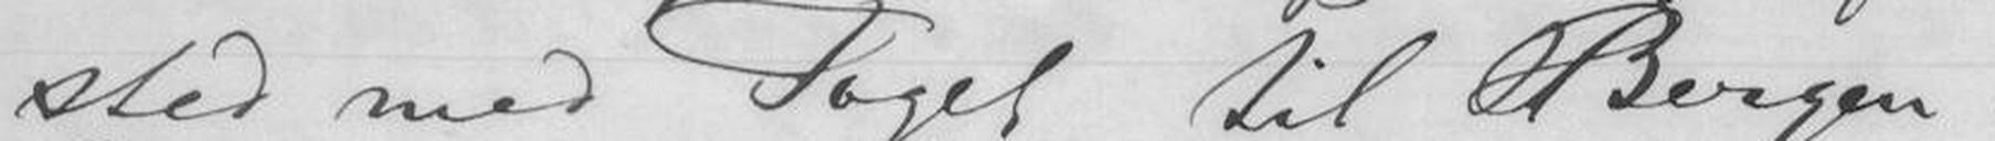sted med Toget til Bergen.
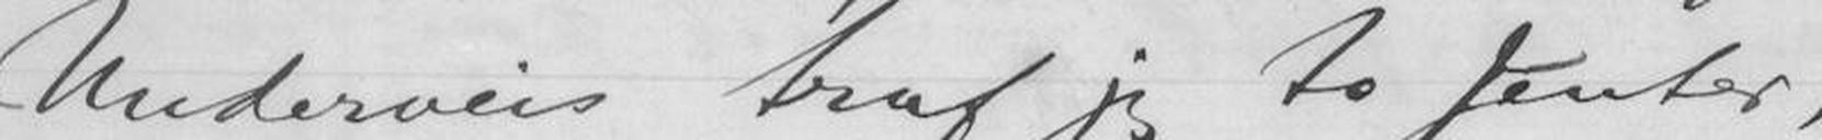Underveis traf jeg to Jenter,
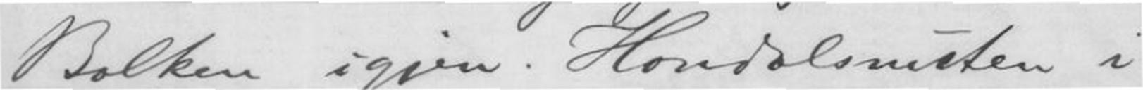Bolken igjen. Hondalsnuten i
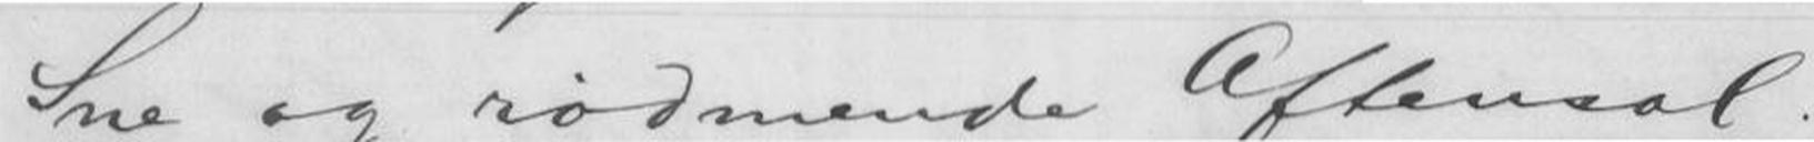Sne og rødmende Aftensol.
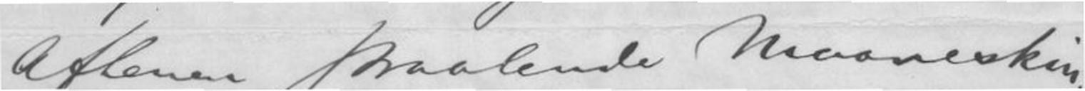Aftenen straalende Maaneskin.
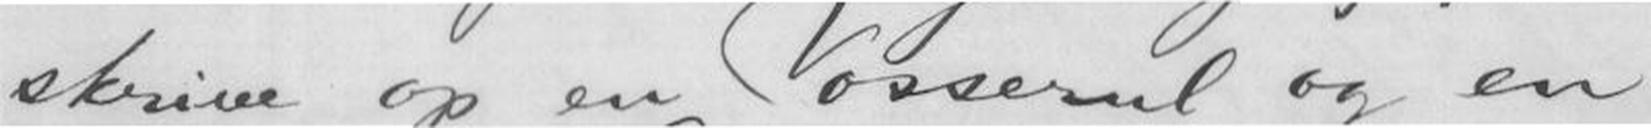skrive op en Vosserul og en
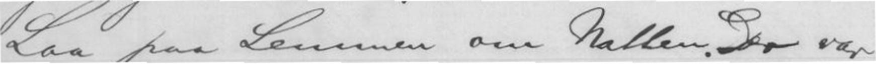Laa paa Lemmen om Natten. Der var
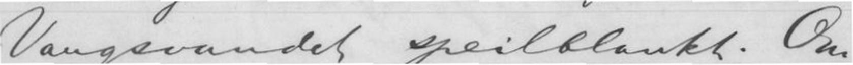Vangsvandet speilblank. Om
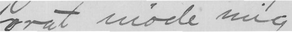forat møde mig,
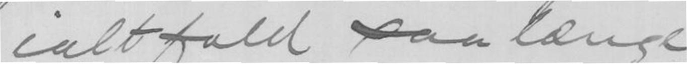ialtfald saa længe
